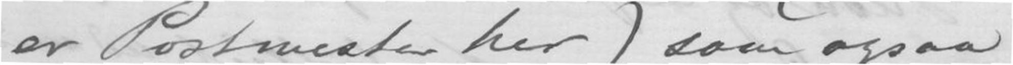er Postmester her) som ogsaa
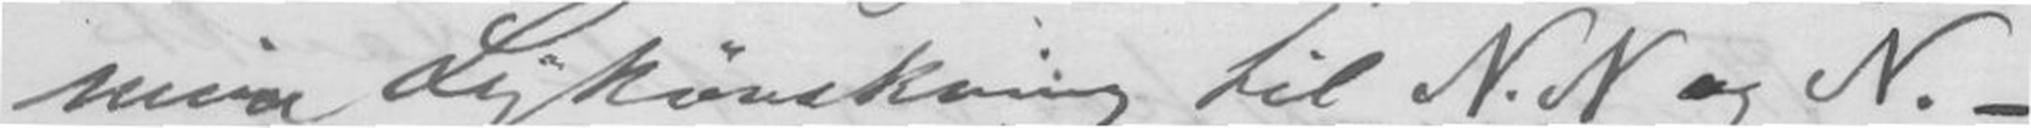min Lykønskning til N. N. og N. -
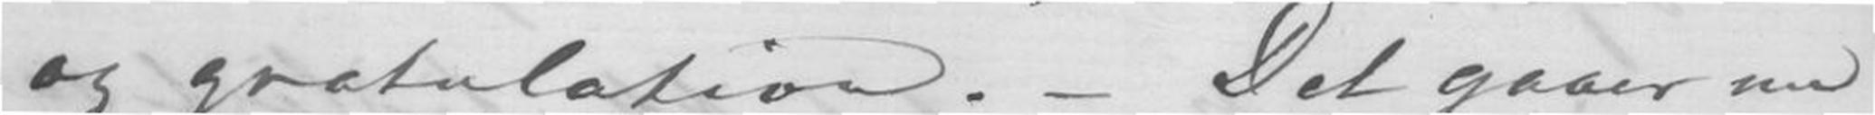og gratulation. - Det gaar nu
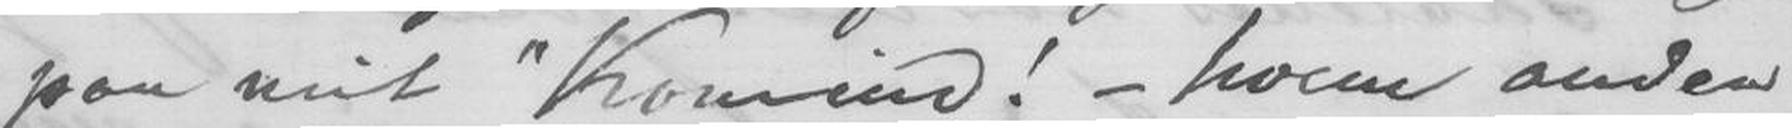paa mit "Komind! - hvem anden
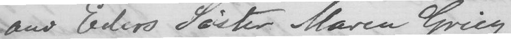end Eders Søster Maren Grieg
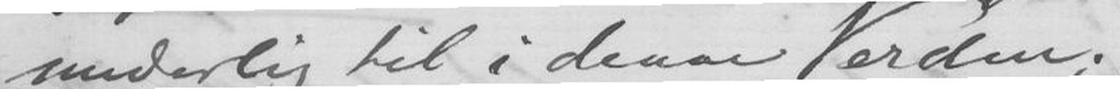underlig til i denne Verden;
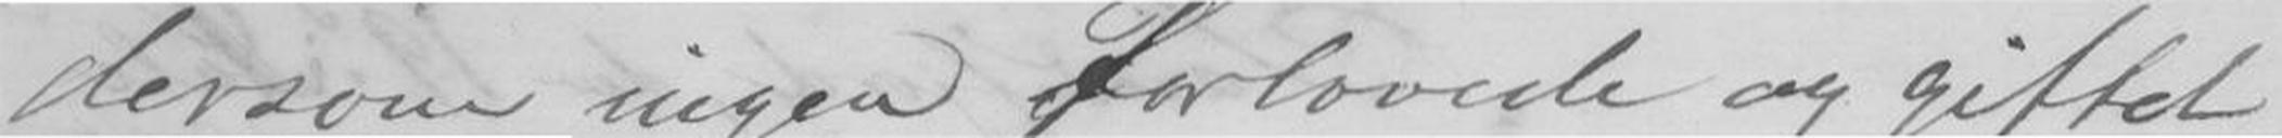dersom ingen Forlovede og giftet
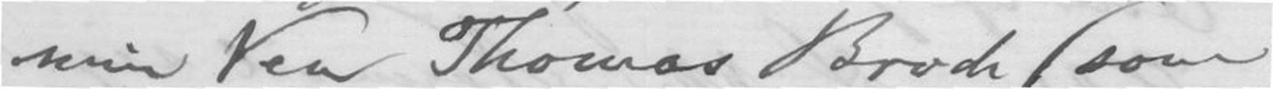min Ven Thomas Broch (som
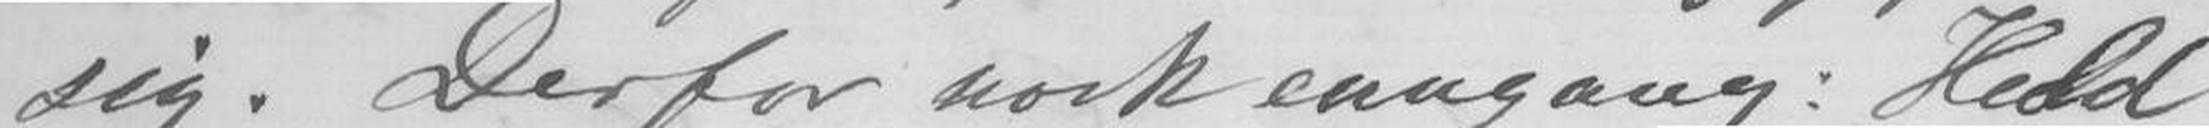sig. Derfor nok enngang: Held
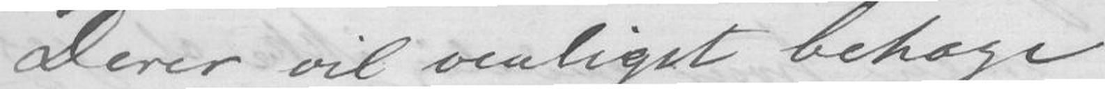Derer vil venligst behage
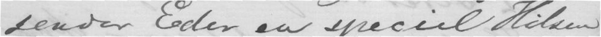sender Eder en speciel Hilsen
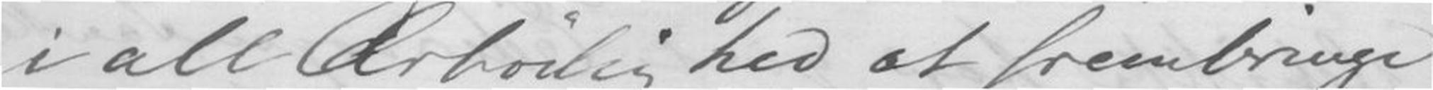i all Ærbødighes at frembringe
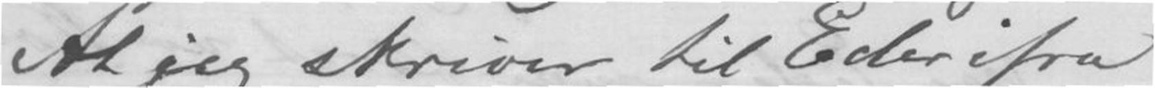At jeg skriver til Eder ifra
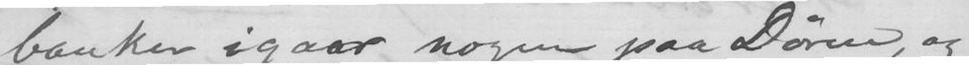banker igaar nogen paa Døren, og
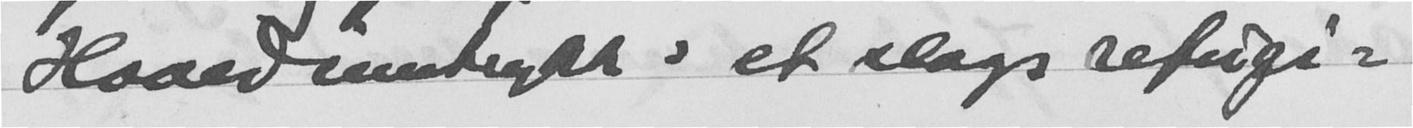Hovedinntrykk: et slags refugi-
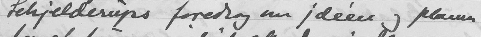Schjelderups foredrag om ideen og planen
